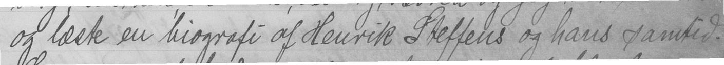og læste en biografi af Henrik Steffens og hans samtid.
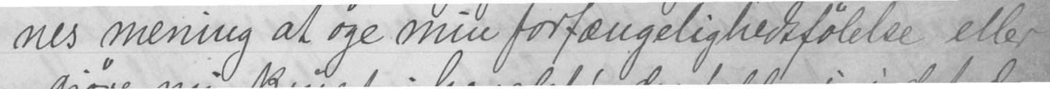nes mening at øge min forfængelighedsfølelse eller
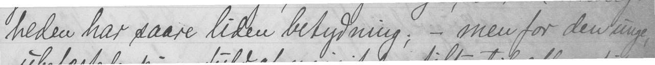heden har saare liden betydning; - men for den unge,
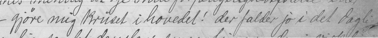- gjøre mig kruset i hovedet! der falder jo i det dagli-
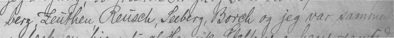berg, Leuthen, Rensch, Seeberg, Broch og jeg var sammen
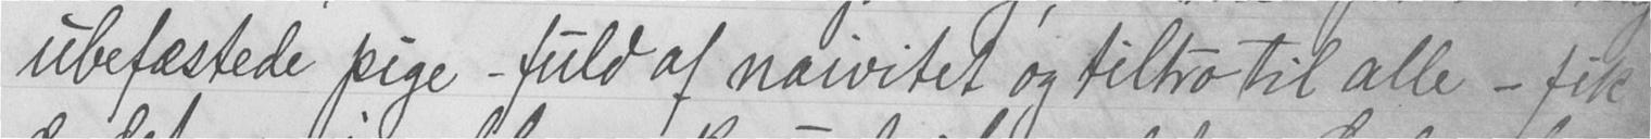ubefæstede pige - fuld af naivitet og tiltro til alle - fik
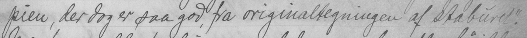pien, der dog er saa god, fra origmnaltegningen af staburet."
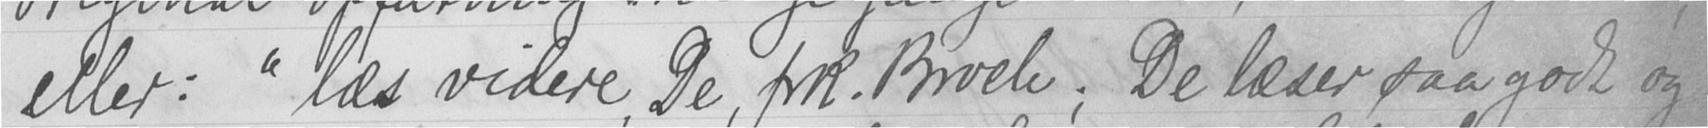eller: "læs videre, De, frk. Broch; De læser saa godt og
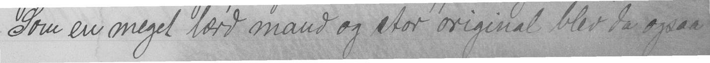Som en meget lærd mand og stor original blev da ogsaa
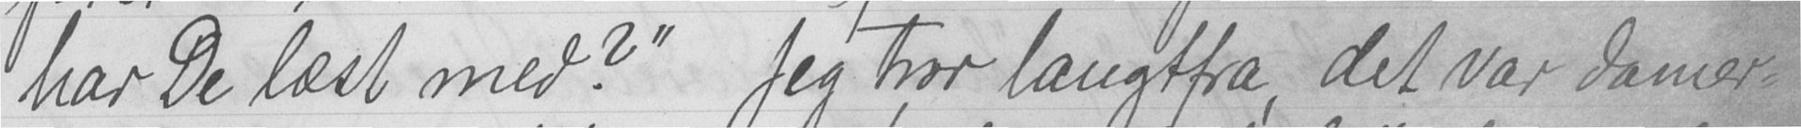har De læst med?" Jeg tror langtfra, det var damer-
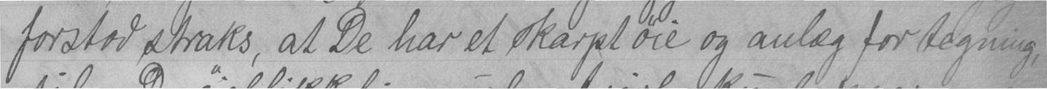forstod straks, at De har et skarpt øie og anlæg for tegning,
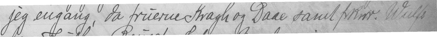jeg engang, da fruerne Kragh og Daae samt frknr. Wulfs-
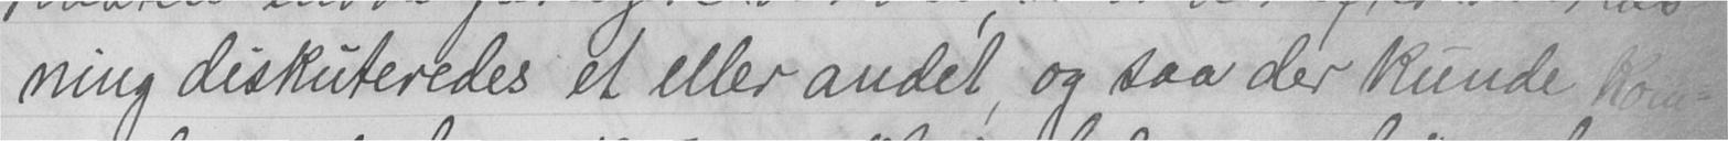ning diskuteredes et eller andet, og saa der kunde kom-
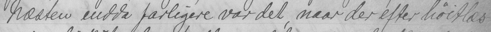Næsten endda farligere var det, naar der efter høitlæs-
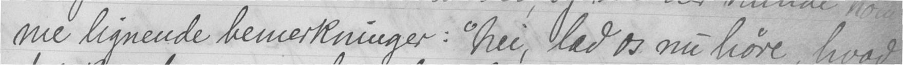me lignende bemerkninger: "Nei, lad os nu høre, hvad
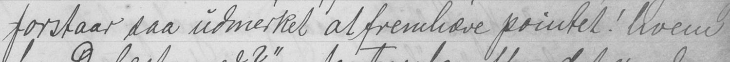forstaar saa udmerket at fremhæve pointet! hvem
